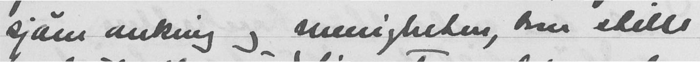sjøen Anking og menigheten, som stille
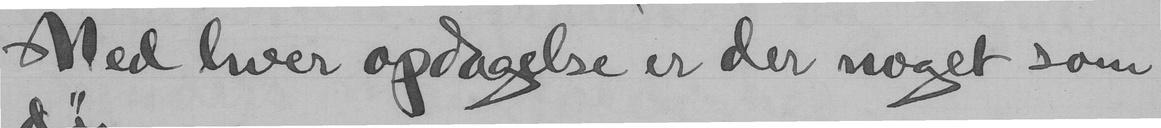Med hver opdagelse er der noget som
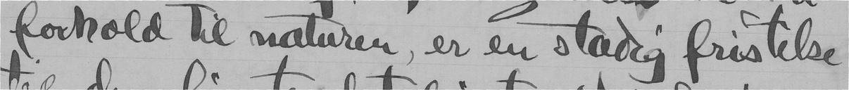forhold til naturen, er en stadig fristelse
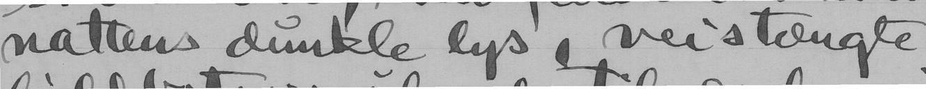nattens dunkle lys, veistængte
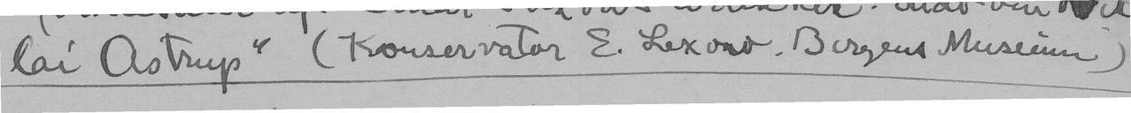lai Astrup" (Konservator E. Lexow. Bergens Museum)
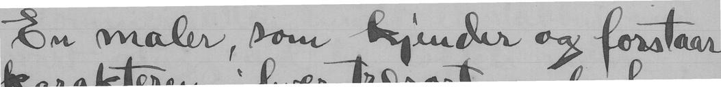En maler, som kjender og forstaar
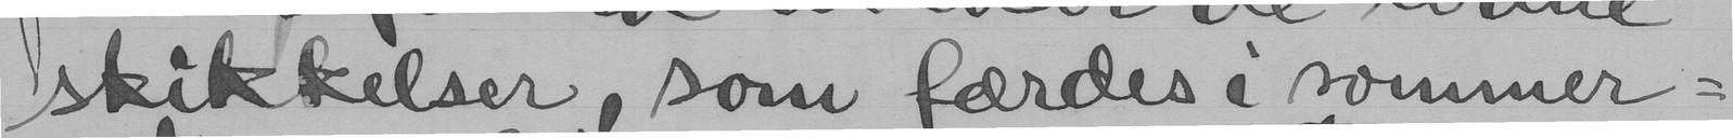skikkelser, som færdes sommer
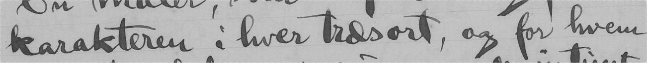karakteren i hver træsort, og for hvem
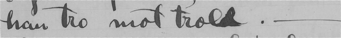han tro mot trold. -
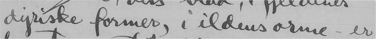dyriske former, i ildens orme - er
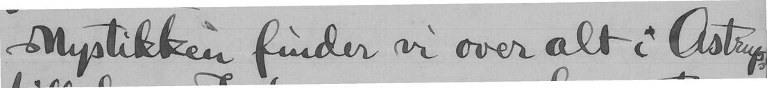Mystikken finder vi over alt i Astrups
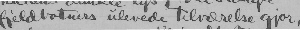fjeldbotners ulevede tilværelse gjør,
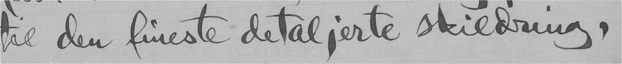til den fineste detaljerte skildring,
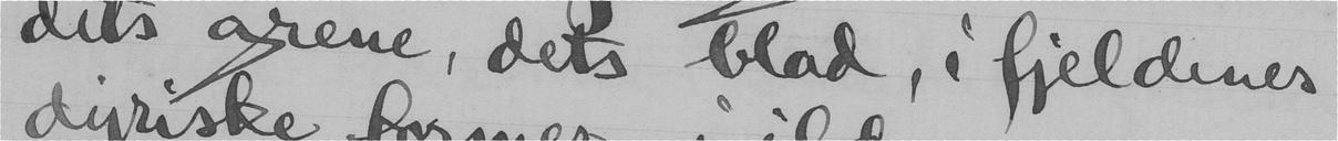dets grene, dets blad, i fieldenes
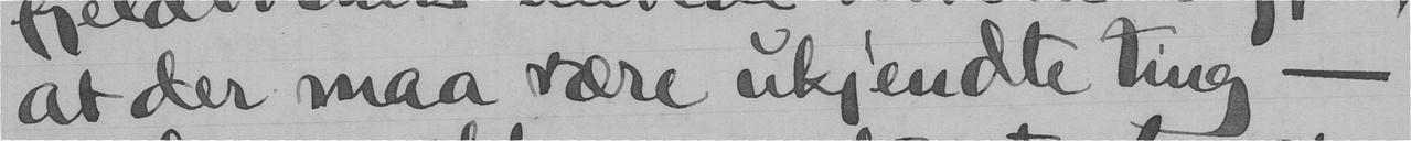at der maa være ukjendte ting -
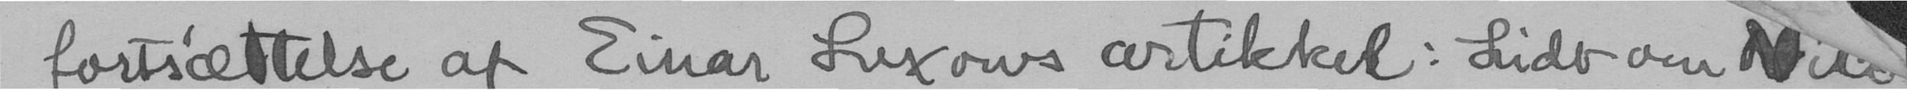fortsættelse af Einar Lexows artikkel: Lidt om Nico-
